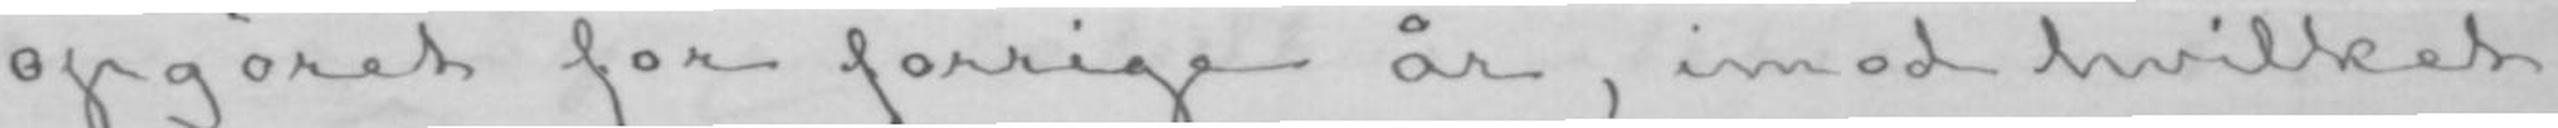opgøret for forrige år, imod hvilket
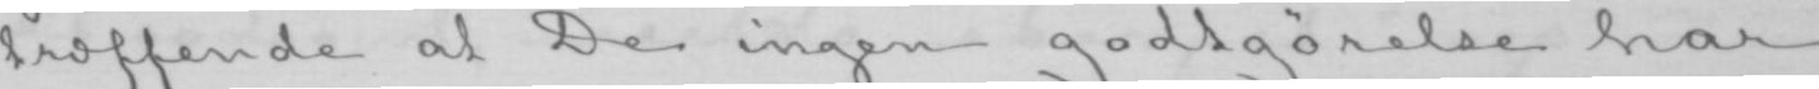træffende at De ingen godtgørelse har
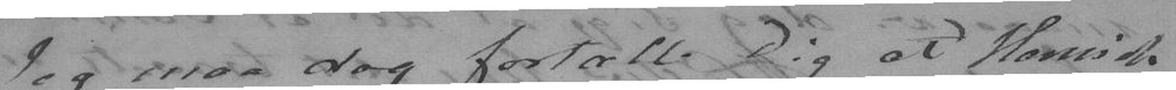Jeg maa dog fortælle Dig at Henrich
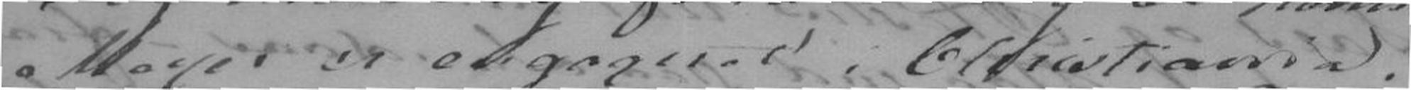Meyer er engageret i Christiania,
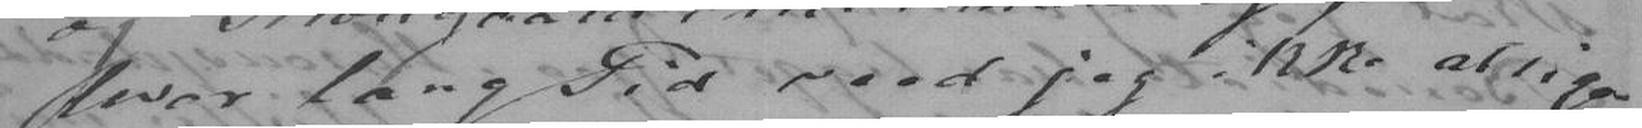hvor lang Tid veed jeg ikke at sige.
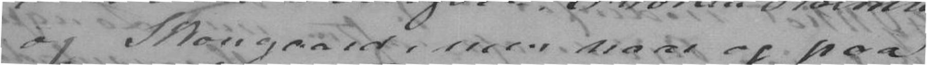og Skougaard, men naar og paa
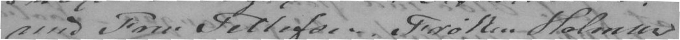med Fru Tellefsen, Frøken Holmers
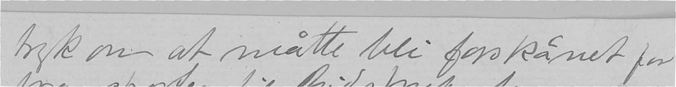tryk om at måtte bli forskånet for
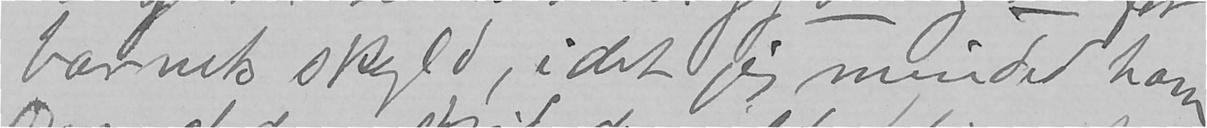barnets skyld, i det jeg minded ham
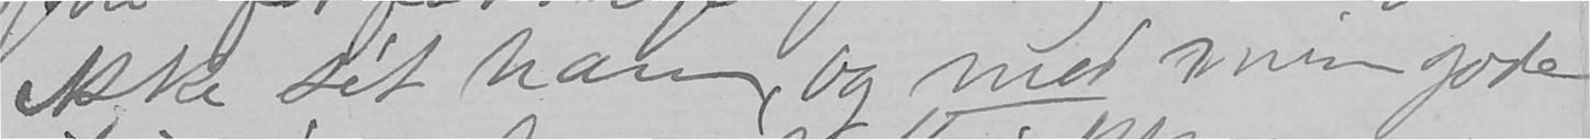ikke sét ham, og med min gode
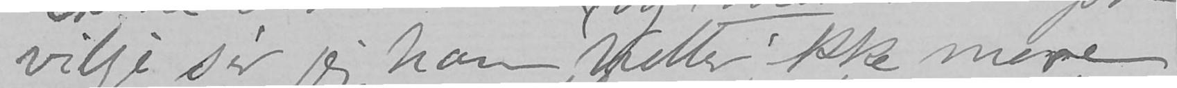vilje sér jeg ham heller ikke mere
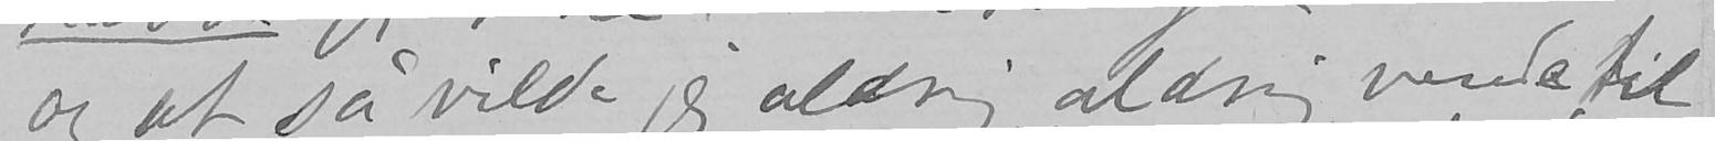og at så vilde jeg aldrig aldrig vende til-
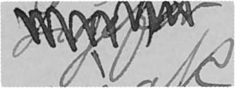ligefør
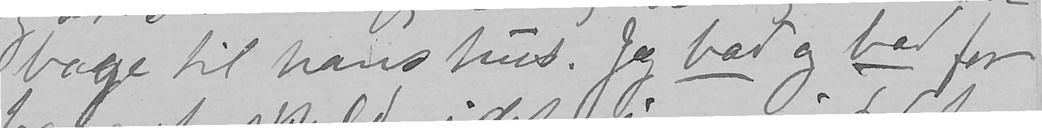bage til hans hus. Jeg bad og bad for
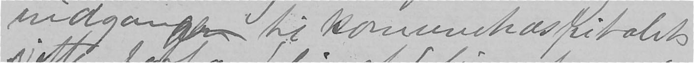indgangen til komunehospitalets
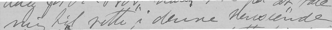mig til rette" i denne henseende.
